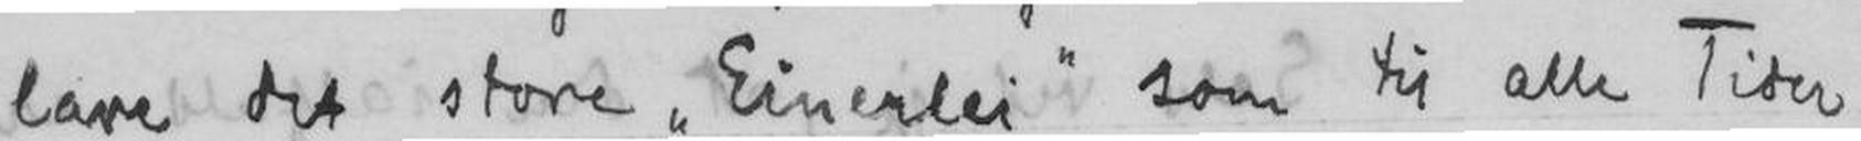lave det store Einerlei som til alle Tider
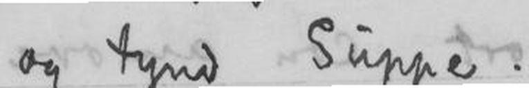og tynd Suppe.
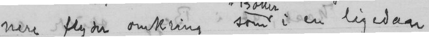nere flyder omkring som i en ligedan
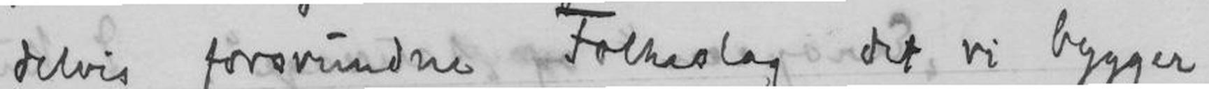delvis forsvundne Folkeslag det vi bygger
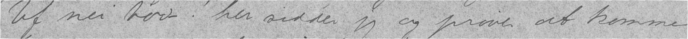Uf nei tøv! her sidder jeg og prøver at komme
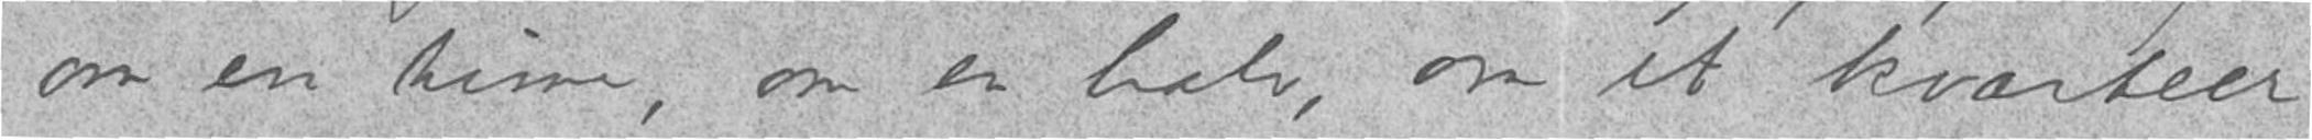om en time, om en halv, om et kvarter —
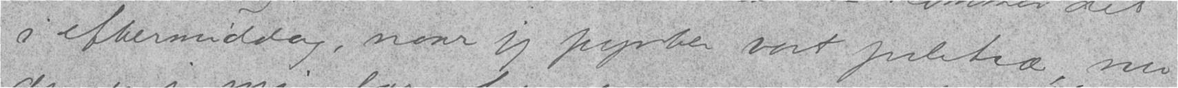i eftermiddag, naar jeg pynter vort juletræ, nu
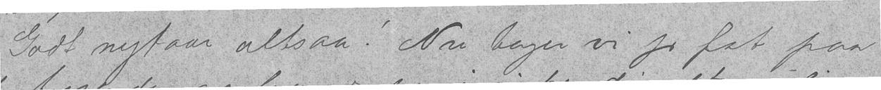Godt nytaar altsaa! Nu tager vi jo fat paa
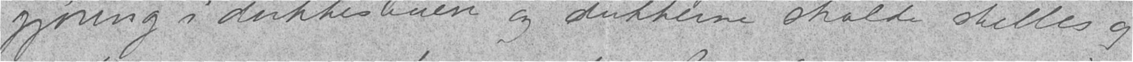gjøring i dukkestuen og dukkerne skulde stelles og
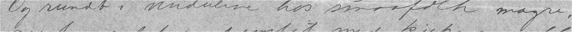Og rundt i vinduerne hos smaafolk magre,
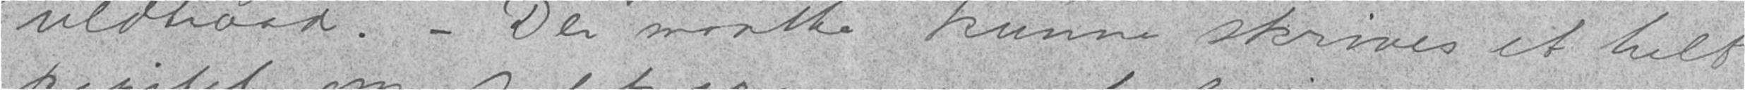uldtraad. — Der maatte kunne skrives et helt
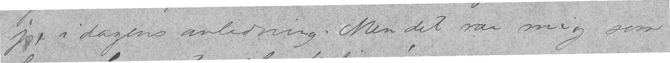jeg, i dagens anledning. Men det var mig som
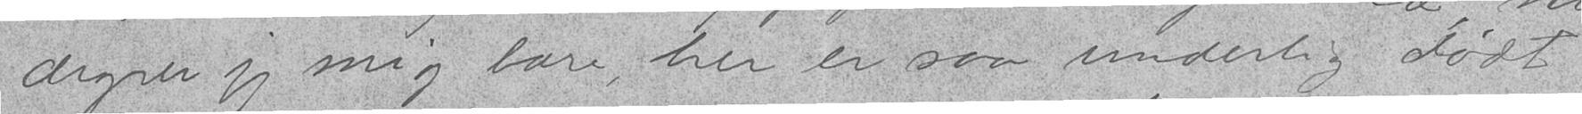ærgrer jeg mig bare, her er saa underlig dødt
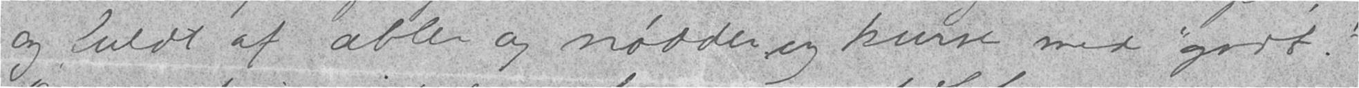og fuldt af æbler og nødder og kurve med "godt".
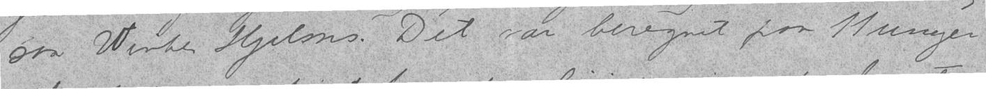saa Winter Hjelms. Det var beregnet paa 11 unger,
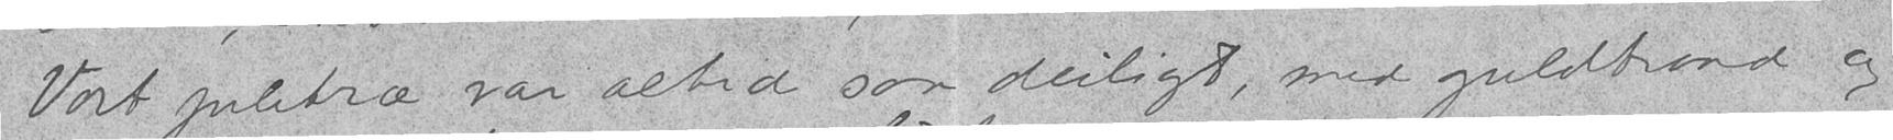Vort juletræ var altid saa deiligt, med guldtraad og
